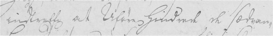indtreffe at Uføre-Hindrede de sædvan-
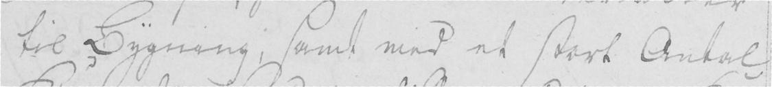til Bygning, samt med et stort Antal
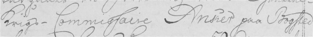Krigs-Commissaire Anker paa Bogsted
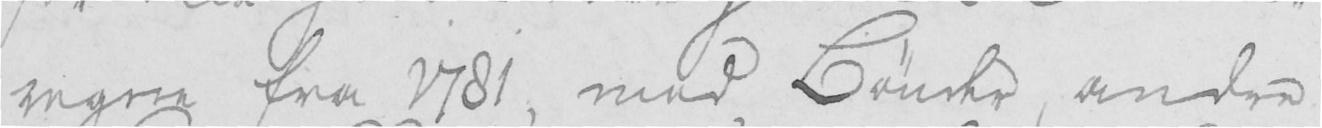regne fra 1781 med Bønder, andre
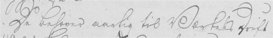De behøver aarlig til Værkets Drift
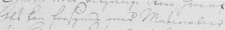det kan forsynes med Materialier
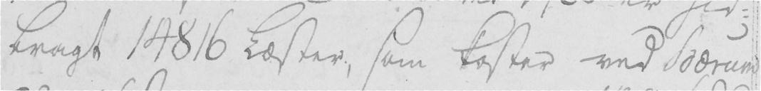bragt 14816 Læster, som koster ved Bærum
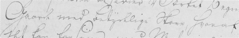Gaarde med betydelige Skove, hvoraf
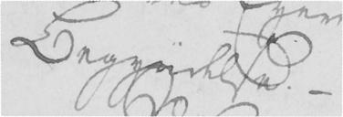Begyndelse. -
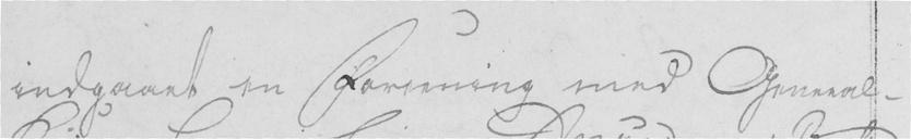indgaaet en Foreening med General
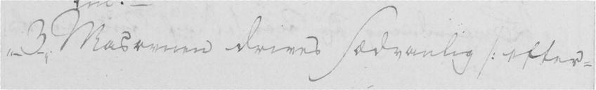"-3-" Masovnen drives sædvanlig /: efter-
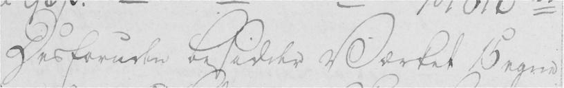Desforuden besidder Værket 15 egne
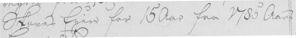Skoves Eyere for 15 Aar fra 1785 Aars
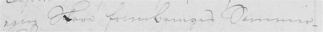egne Skove frembringes Sommer-
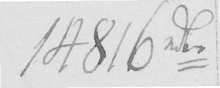14816 Lr
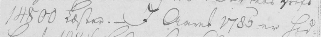14800 Læster. - I Aaret 1785 er hid-
